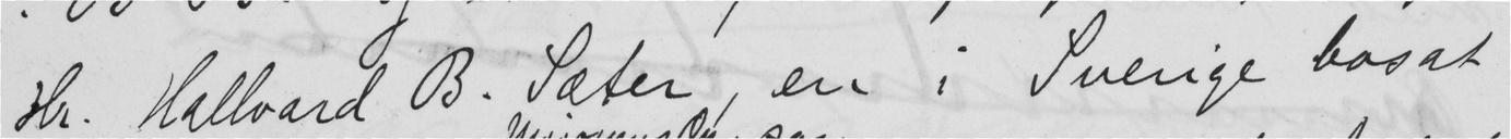Hr. Hallvard B. Sæter en i Sverige bosat
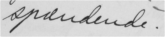spændende.
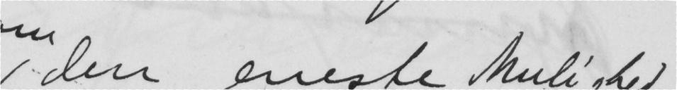den eneste Mulighed
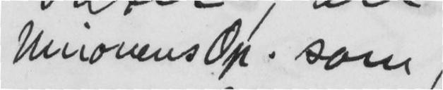UnionsOp. som
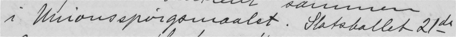i Unionsspørgsmaalet. Slotsballet 21de
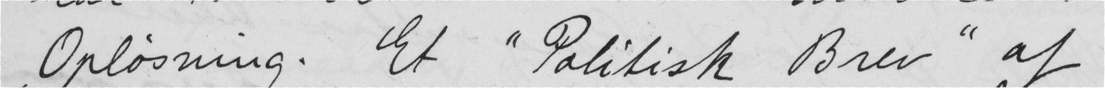Opløsning. Et "Politisk Brev" af
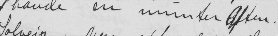havde en munter Aften.
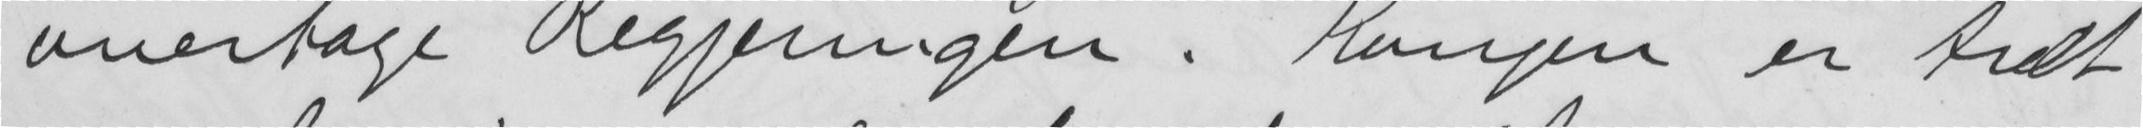overtage Regjeringen. Kongen er træt
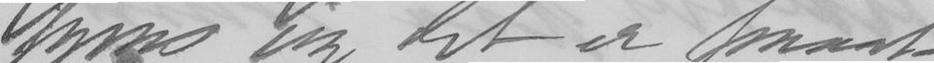synes jeg det er smaat
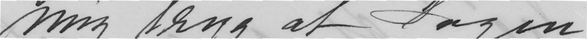mig tryg at Sagen
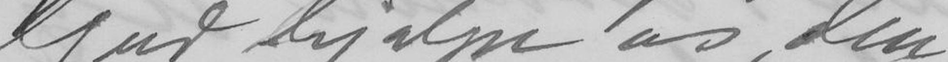Gud hjælpe os, den
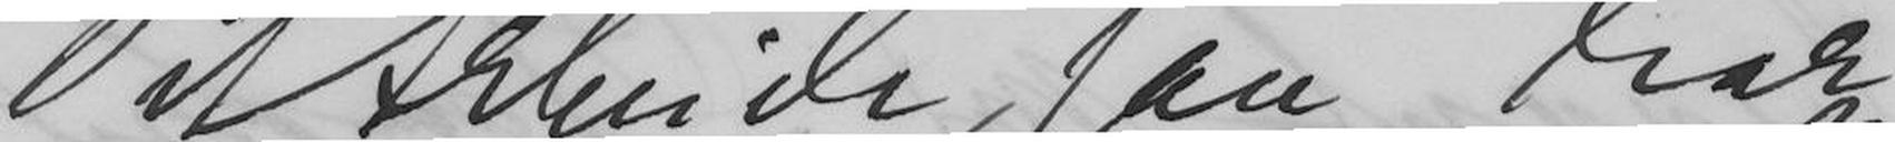sit Arbeide, saa har
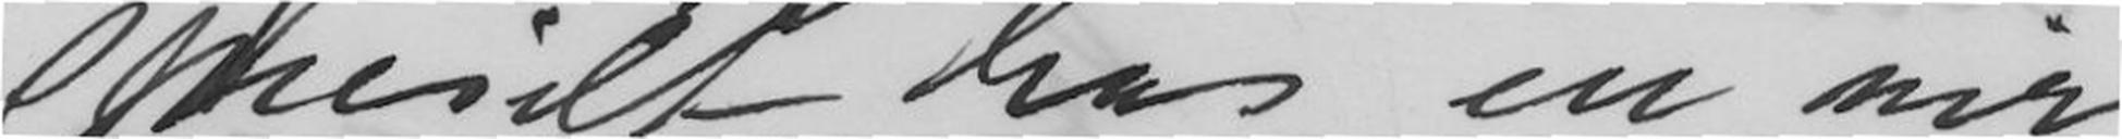spicielt hos en vir-
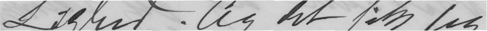Lighed. Og det fik jeg
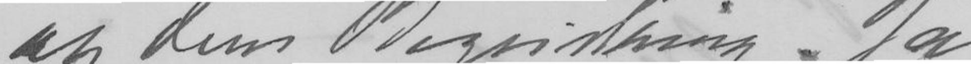og dens Begeistring. Ja
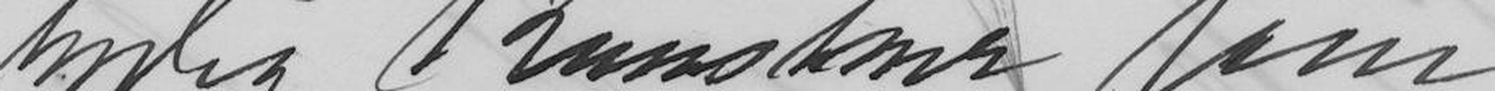kelig Kunstner som
